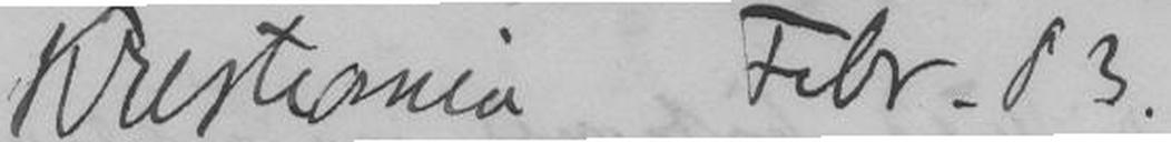Kristiania Febr. 83.
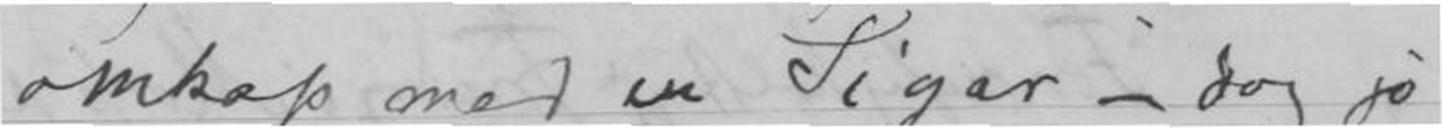omkap med en Sigar - dog jo,
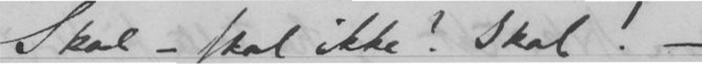Skal - skal ikke? Skal! -
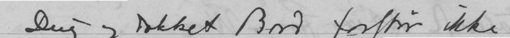Dug og dekket Bord forstår ikke
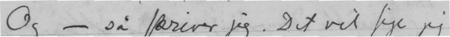Og - så skriver jeg. Det vil sige jeg
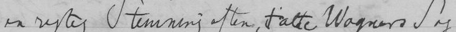en rigtig Stemning aften, talte Wagner Sag,
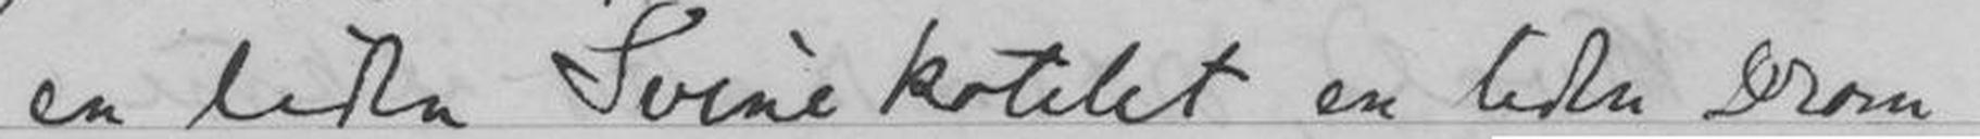en liten Svinekotelet en liden Dram
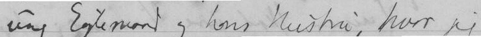ung Egtemand og hans Hustru, hvor jeg
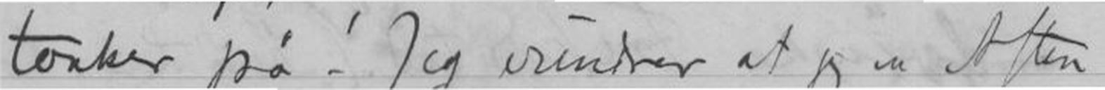tænker på! Jeg erindrer at jeg en Aften,
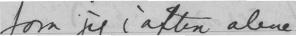som jeg i aften alene
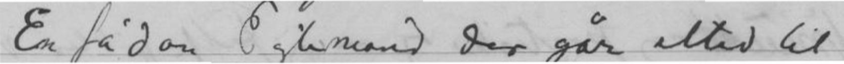En sådan Egtemand går eltid til
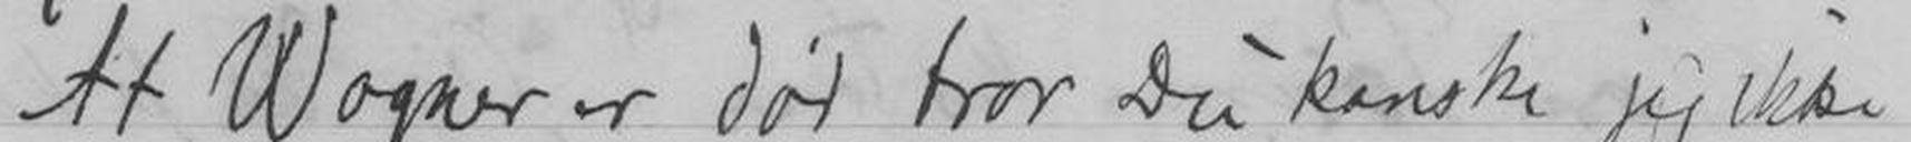At Wagner er død tror Du kanske jeg ikke
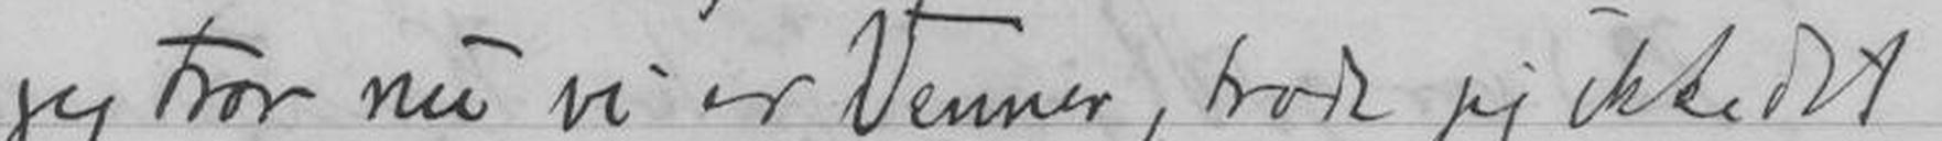jeg tror nu vi er Venner, trode jeg ikke det
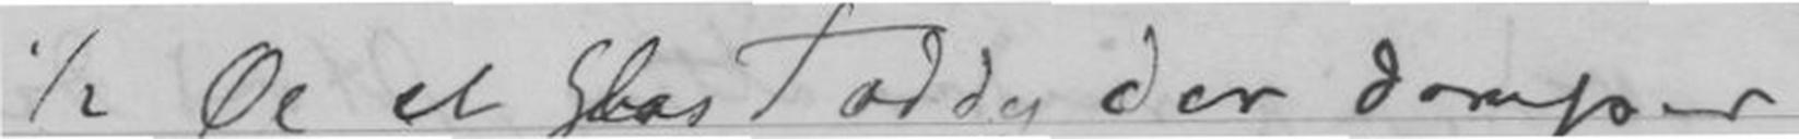½ Øl et Glas Toddy der damper
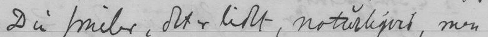Du smiler, det er lidt, naturligvis, men
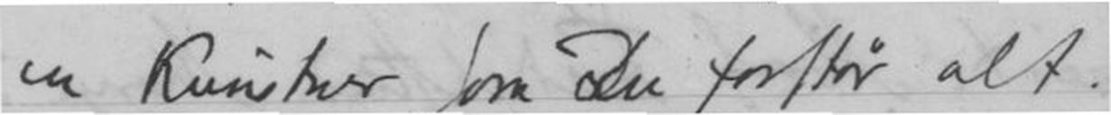en Kunstner som Du forstår alt!
